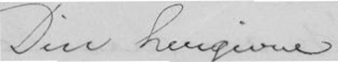Din hengivne
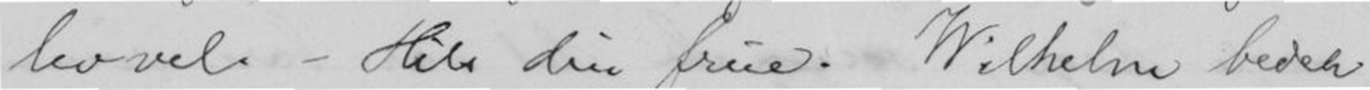levvel. - Hils din frue. Wilhelm beder
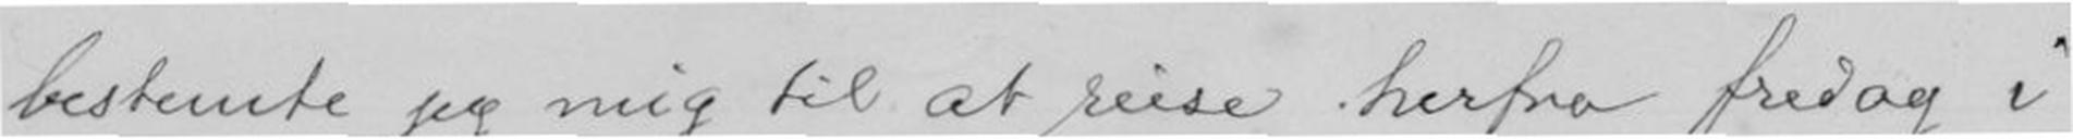bestemte jeg mig til at reise herfra fredag i
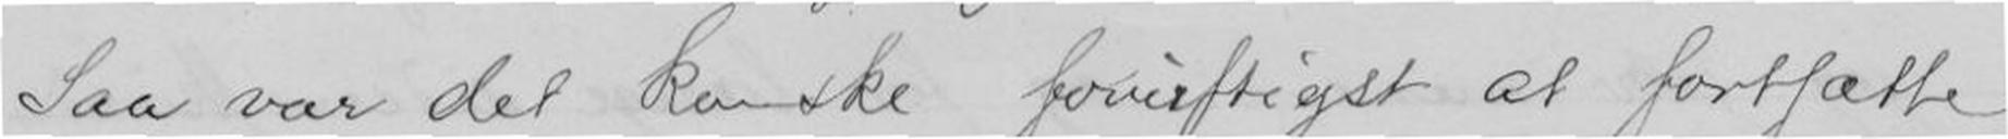Saa var det kanske fornuftigst at fortsætte
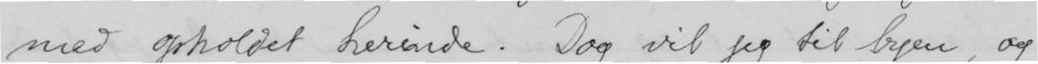med opholdet herinde. Dog vil jeg til byen, og
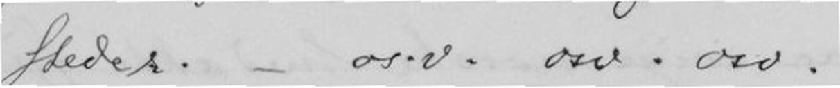steder. - os.v. osv. osv. -
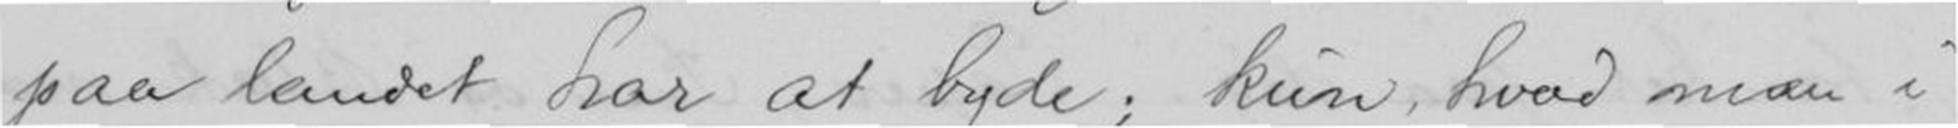paa landet har at byde; kun hvad man i
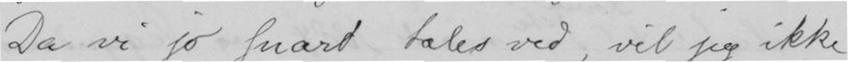Da vi jo snart taler ved, vil jeg ikke
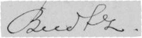Budtz
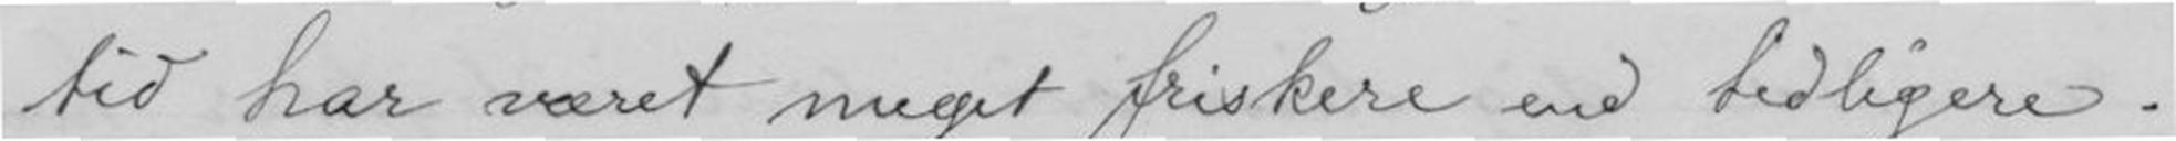tid har været meget friskere end tidligere.
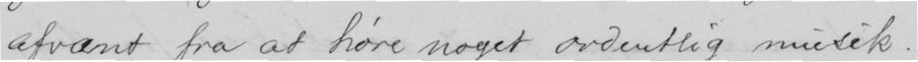afvænt fra at høre noget ordentlig musik.
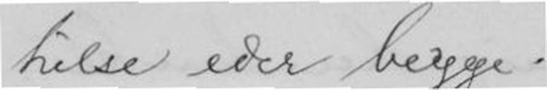hilse eder begge.
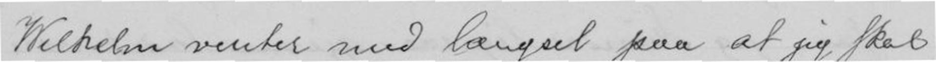Wilhelm venter med længsel paa at jeg skal
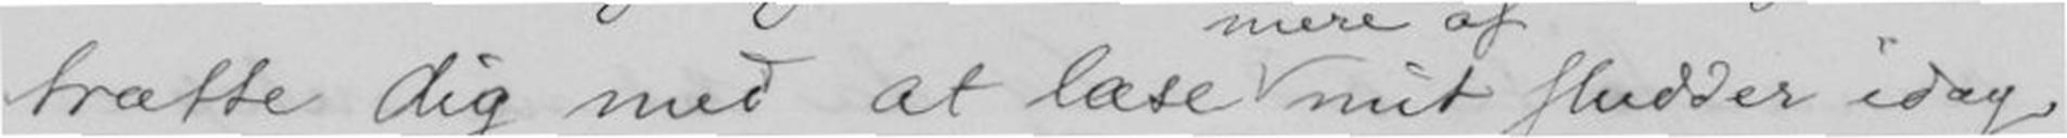trætte dig med at læse mit sludder idag.
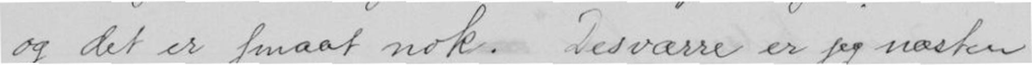- og det er smaat nok. Desværre er jeg næsten
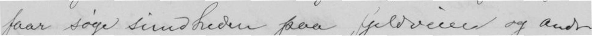faar søge sundheden paa fjeldveien og andre
